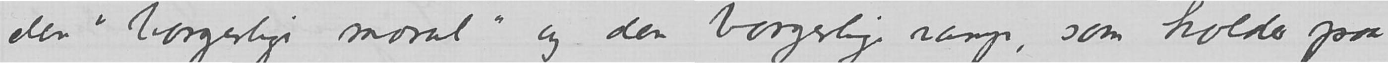ut over den «borgerlige moral» og den borgerlige ramp, som holder paa
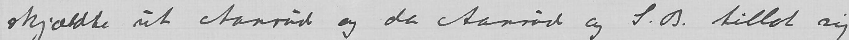skjældte ut Aanrud og da Aanrud og S.B. tillot sig
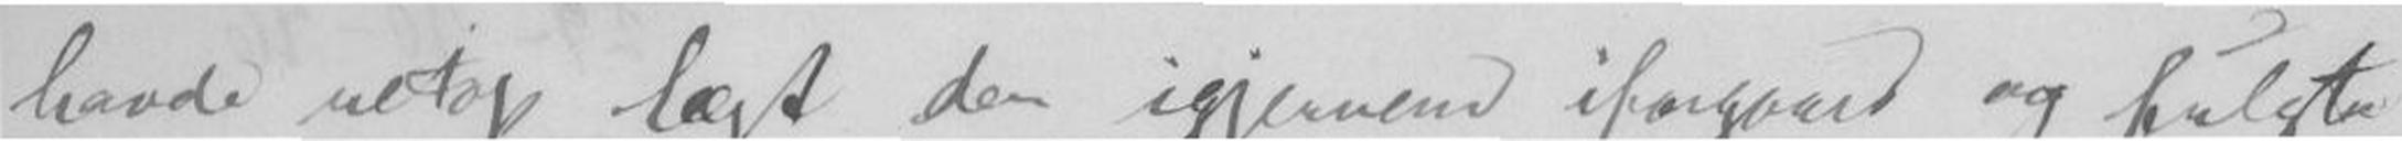havde netop læst den igjennem iforgaars og fulgte
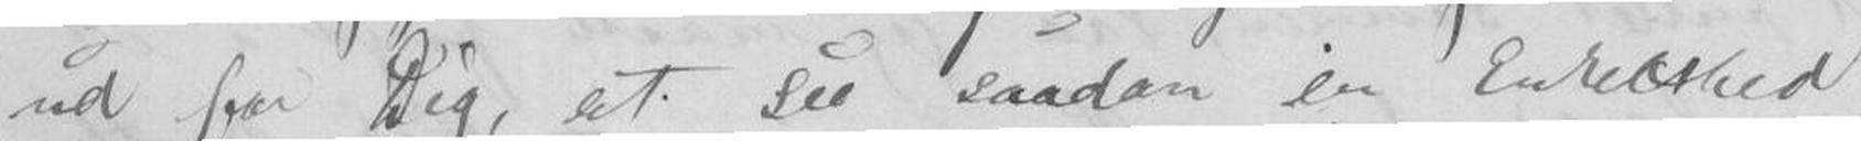ud for Dig, at see saadan en Enkelthed
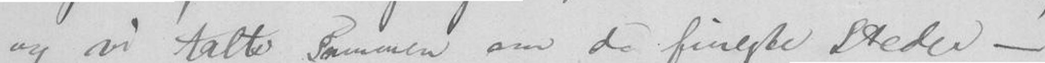og vi talte sammen om de fineste steder -
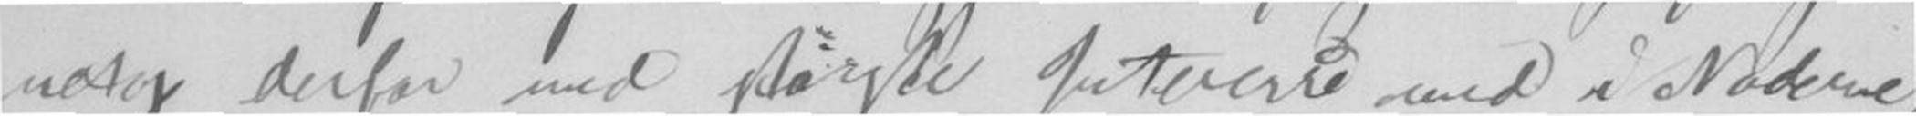netop derfor med største Interesse med i Noderne,
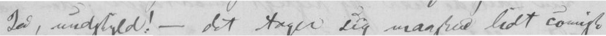Ja, undskyld! - det tager sig maaskee lidt comiskt
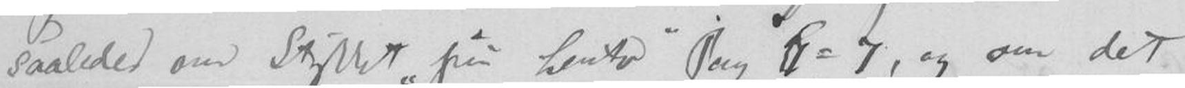saaledes om Stykket po Certo Pag 6=7, og om det
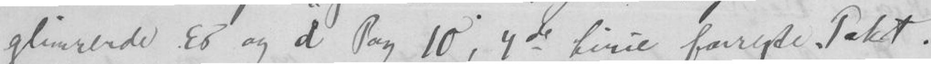glimrende Es og d Pag 10, 4de linie første Takt.
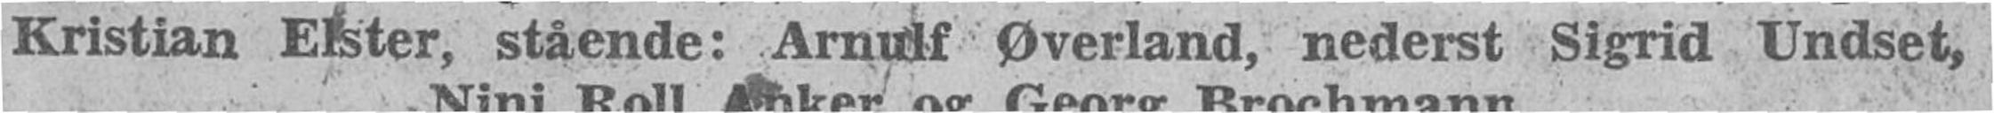Kristian Elster, stående: Arnulf Øverland, nederst Sigrid Undset,
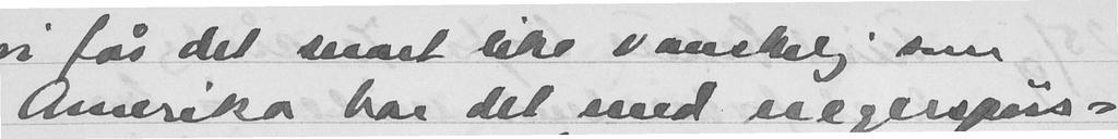Amerika har det med negerspørs-
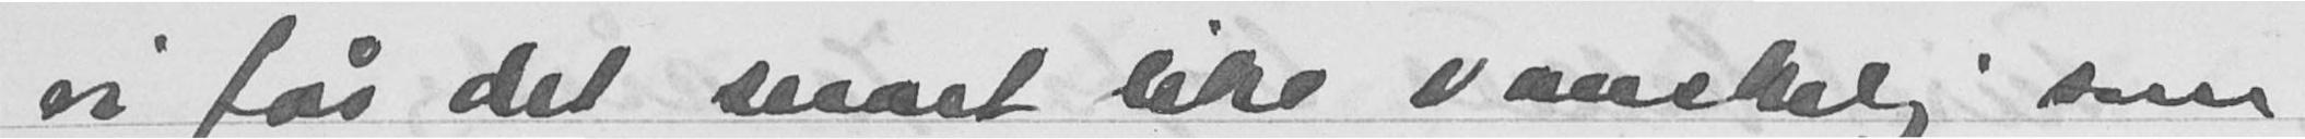vi får det snart like vanskelig som
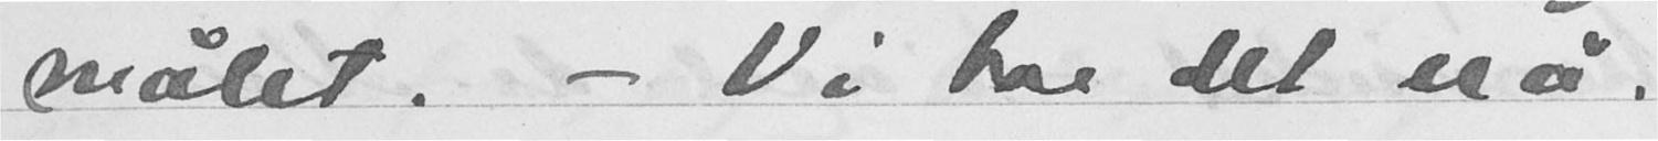målet. - Vi har det nå. -
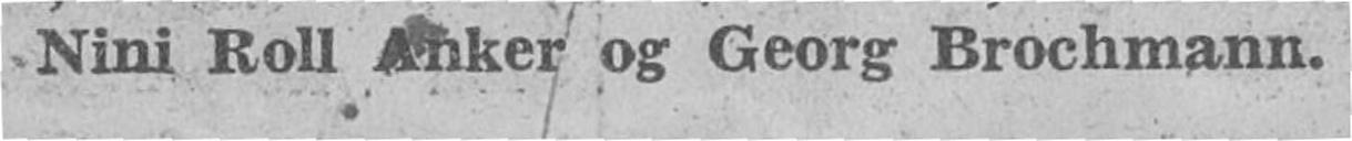Nini Roll Anker og Georg Brochmann.
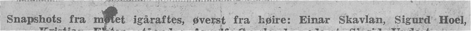Snapshots fra møtet igåraftes, øverst fra høire: Einar Skavlan, Sigurd Hoel,
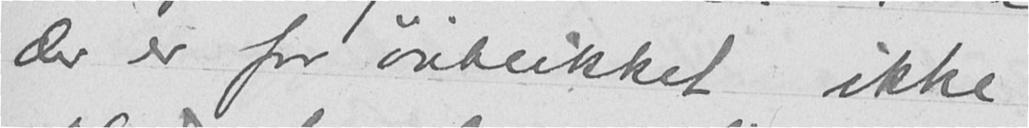der er for øieblikket ikke
